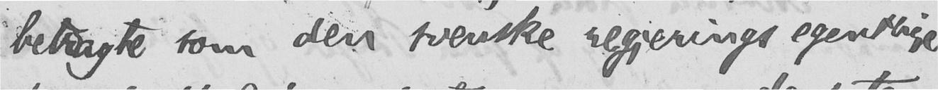betragte som den svenske regjerings egentlige
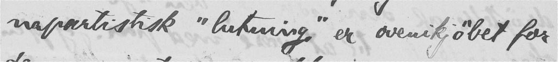napartistisk "lutning" er ovenikjøbet for
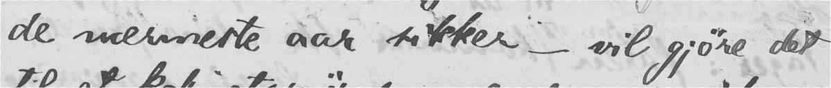de nærmeste aar sikker – vil gjøre det
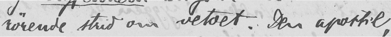rørende strid om vetoet. Den apostil
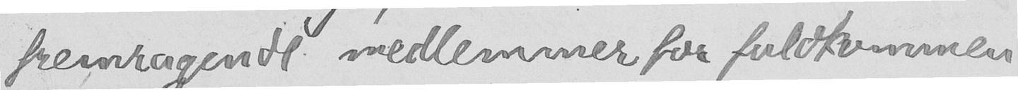fremragende medlemmer for fuldkommen
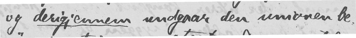og derigjennem undgaar den unionen be-
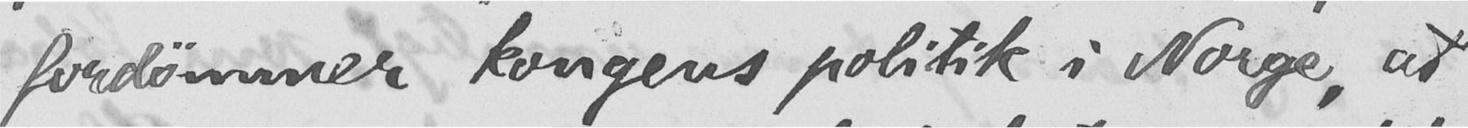fordømmer kongens politik i Norge, at
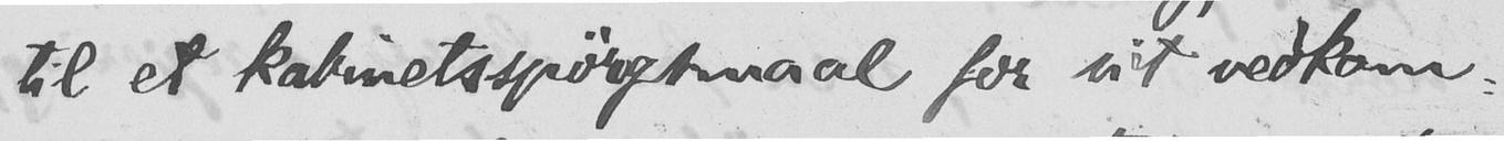til et kabinetsspørgsmaal for sit vedkom-
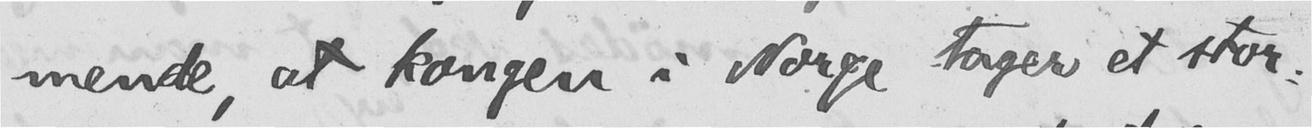mende, at kongen i Norge tager et stor-
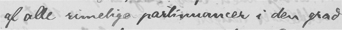af alle rimelige partinuancer i den grad
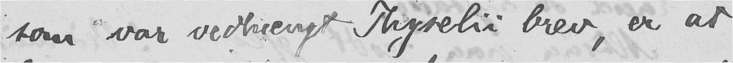som var vedhængt Thyselii brev, er at
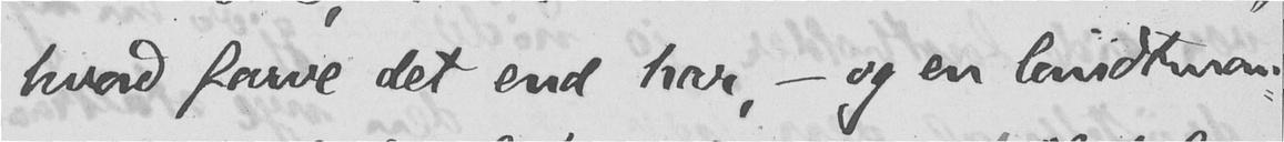hvad farve det end har – og en landtman-
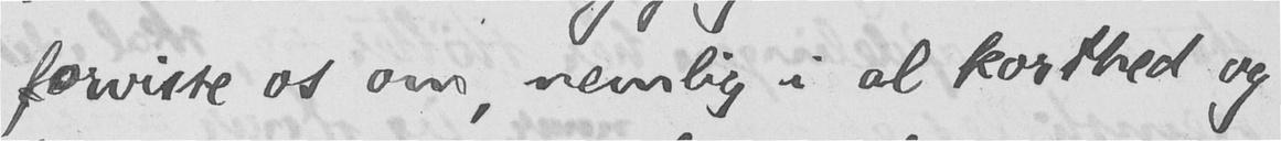forvisse os om, nemlig i al korthed og
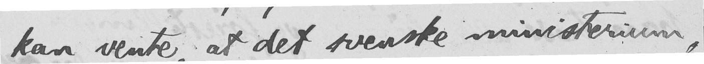kan vente, at det svenske ministerium,
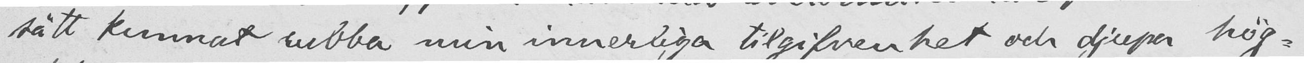sätt kunnat rubba min innerliga tilgifvenhet och djupa høg-
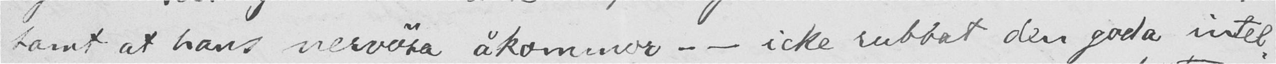samt at hans nervøsa åkommor - - icke rubbat den goda intel-
